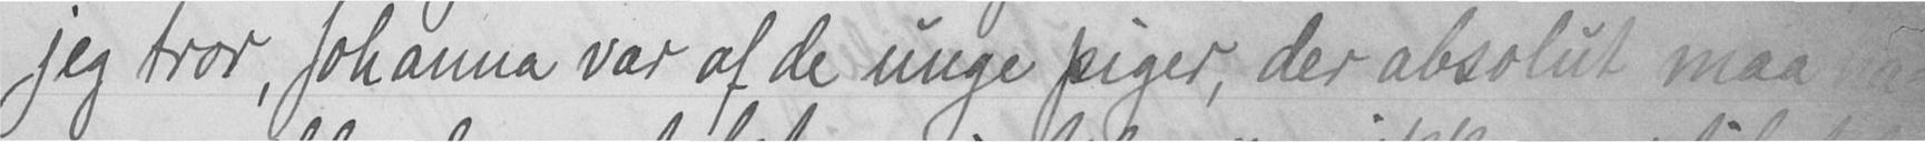jeg tror, Johanna var af de unge piger, der absolut maa ha-
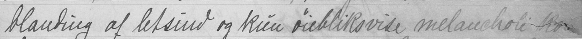blanding af letsind og kun øiebliksvise melancholi kom
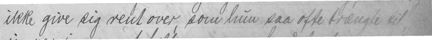ikke give sig rent over, som hun saa ofte trængte til, -
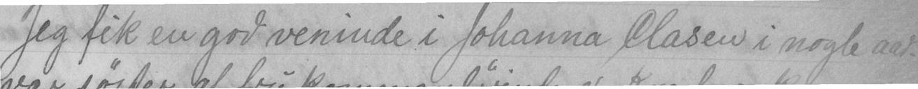Jeg fik en god veninde i Johanna Clasen i nogle aar.
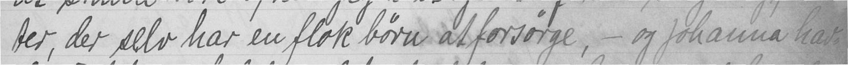ter, der selv har en flok børn at forsørge, - og Johanna had-
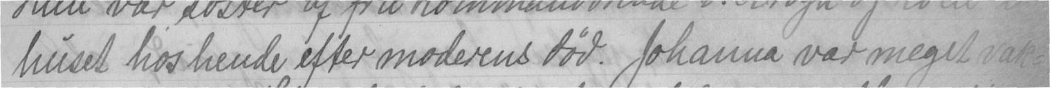huset hos hende efter moderens død. Johanna var meget vak-
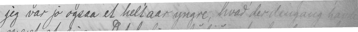jeg var jo ogsaa et helt aar yngre, hvad der dengang havde
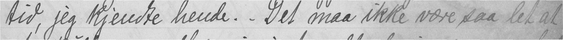tid, jeg kjendte hende. - Det maa ikke være saa let at
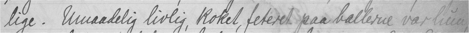lige. Umaadelig livlig, koket, feteret paa ballerne var hun,
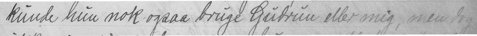kunde hun nok ogsaa bruge Gudrun eller mig, men dog
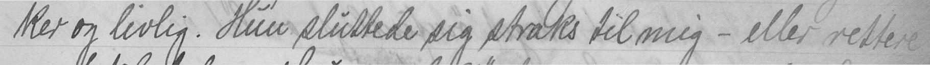ker og livlig. Hun sluttede sig straks til mig - eller rettere
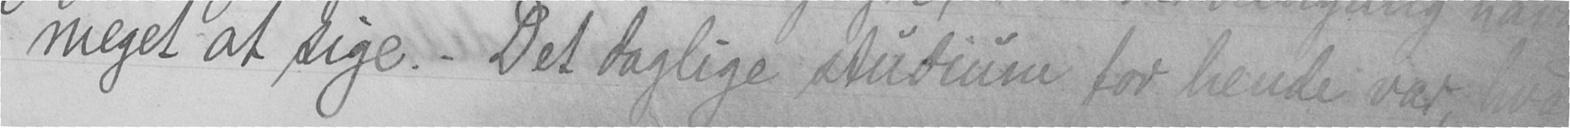meget at sige. - Det daglige studium for hende var, hva
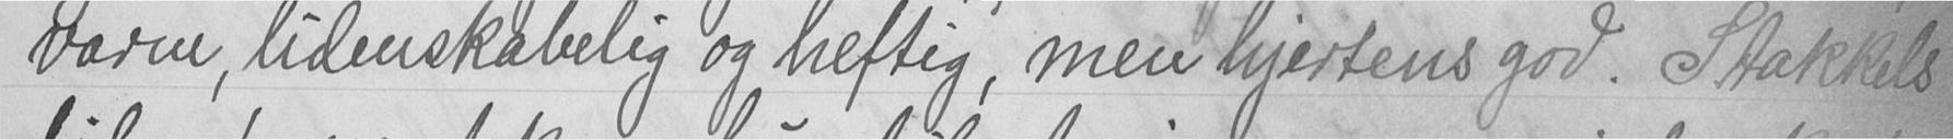varm, lidenskabelig og heftig, men hjertens god. Stakkels
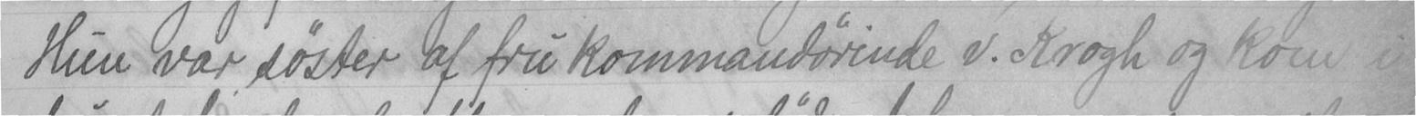Hun var søster af fru kommandørinde v. Krogh og kom i
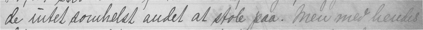de intet somhelst andet at stole paa. Men med hendes
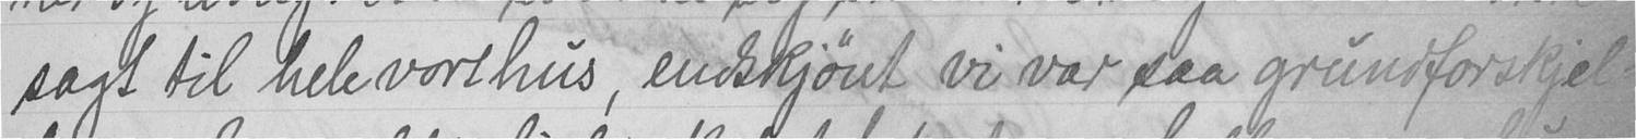sagt til hele vort hus, endskjønt vi var saa grundforskjel-
